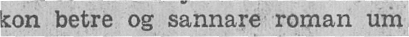kon betre og sannare roman um
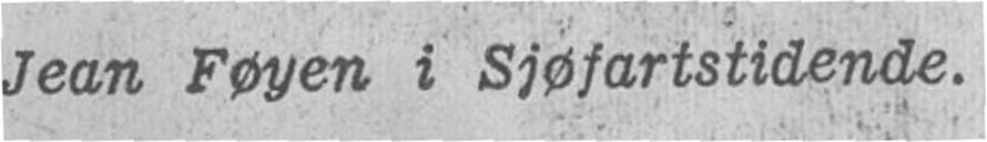Jean Føyen i Sjøfartstidende.
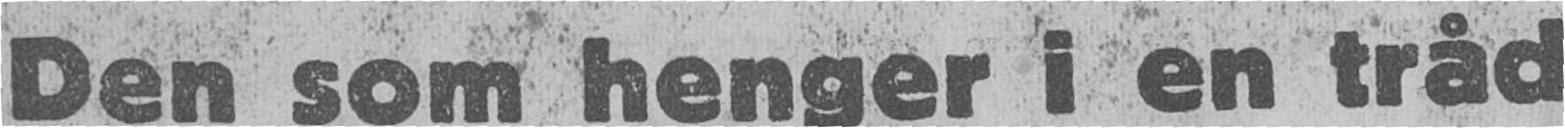Den som henger i en tråd
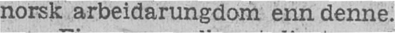norsk arbeidarungdom enn denne.
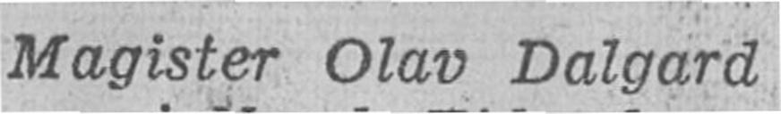Magister Olav Dalgard
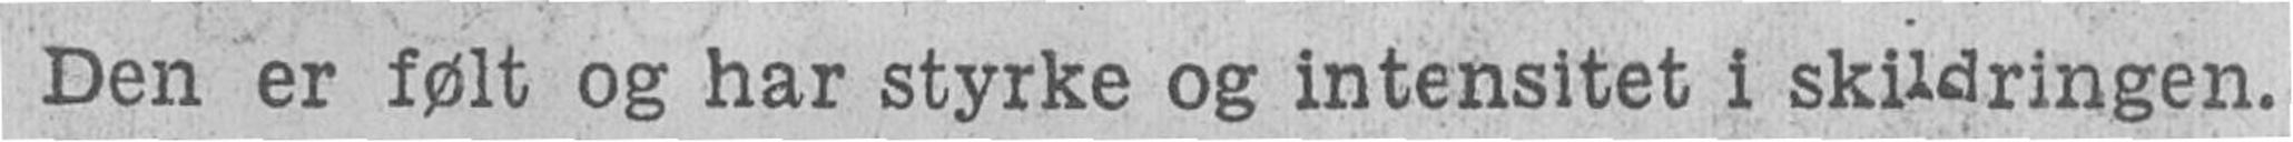Den er følt og har styrke og intensitet i skildringen.
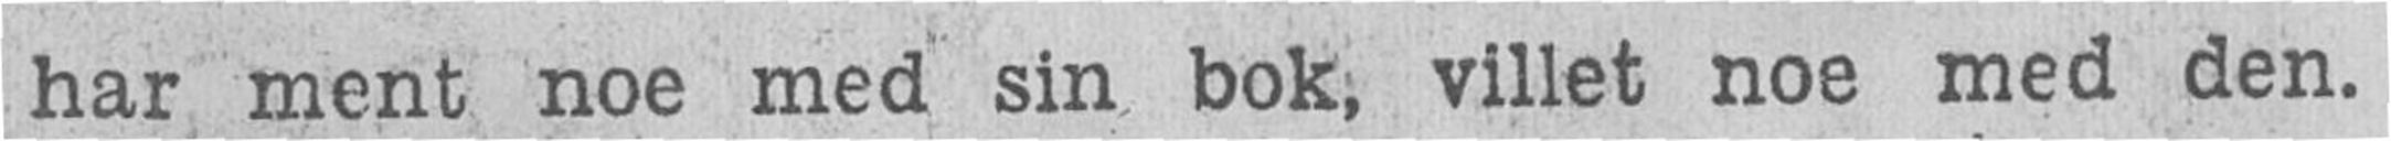har ment noe med sin bok, villet noe med den.
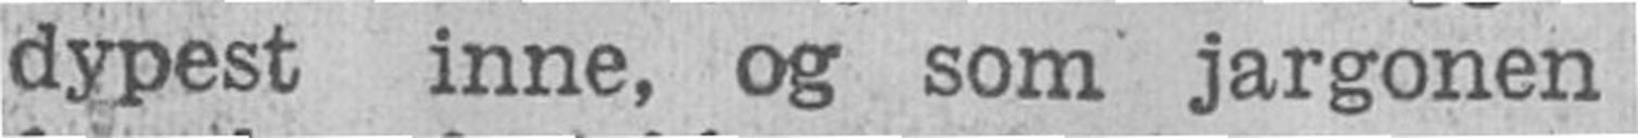dypest inne, og som jargonen
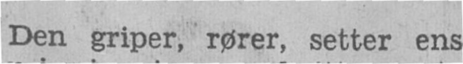Den griper, rører, setter ens
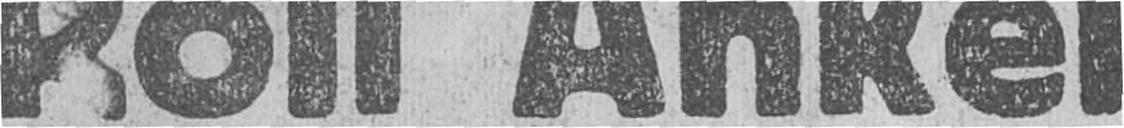Roll Anker
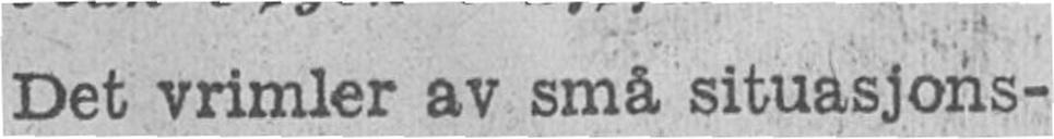Det vrimler av små situasjons-
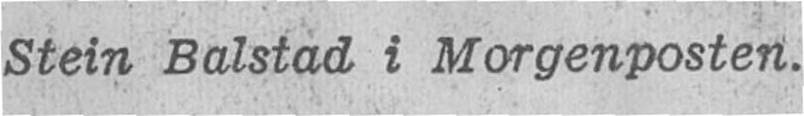Stein Balstad i Morgenposten.
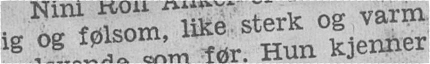ig og følsom, like sterk og varm
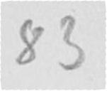83
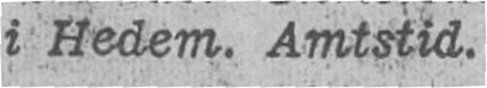i Hedem. Amtstid.
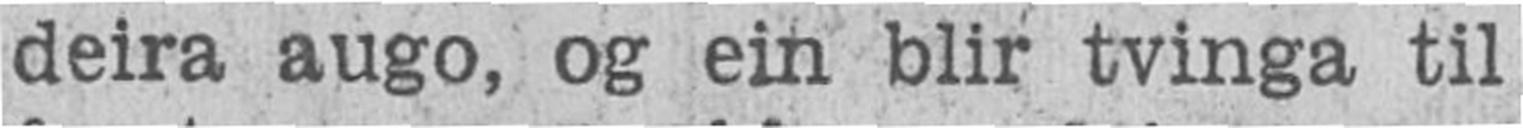deira augo, og ein blir tvinga til
